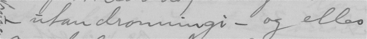– utan dronningi – og elles
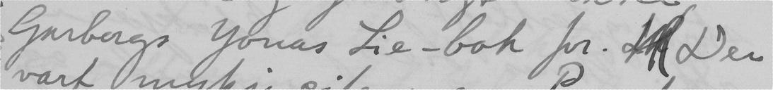Garborgs Jonas Lie-bok for.  Den
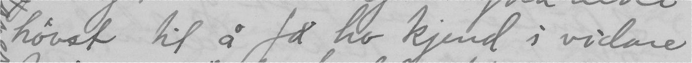hövet til å få ho kjend i vidare
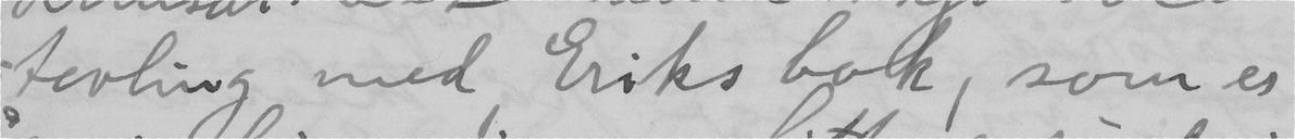tevling med Eriks bok, som er
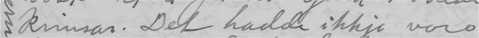krinsar. Det hadde ikkje vore
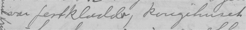var festklædd, Kongehuset
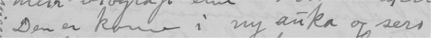Den er kome i ny auka og sers
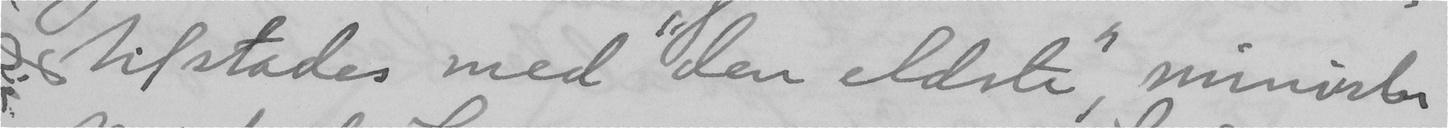tilstades med "den eldste", minister
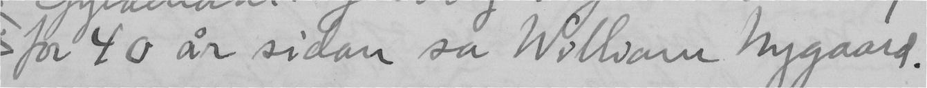for 40 år sidan sa William Nygaard.
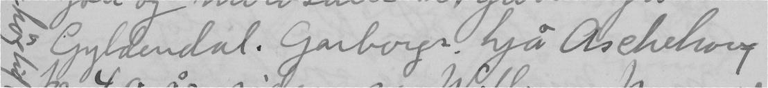Gyldendal. Garborgs hjå Aschehoug
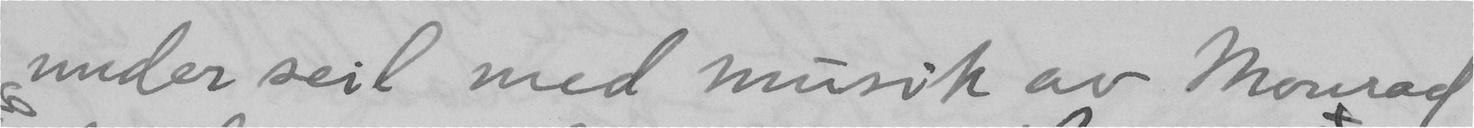under seil med musik av Monrad
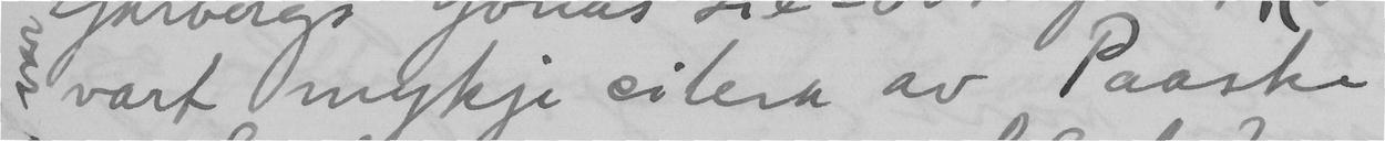vart mykje citera av Paaske
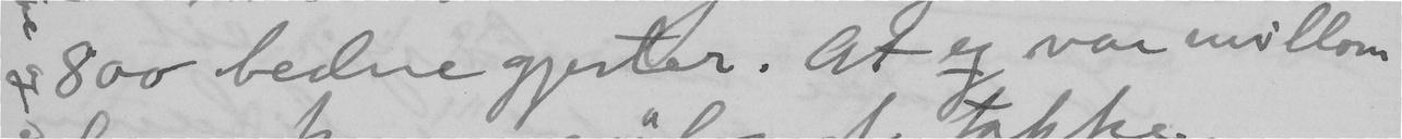800 bedne gjester. At eg var millom
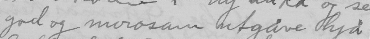god og morosom utgåve hjå
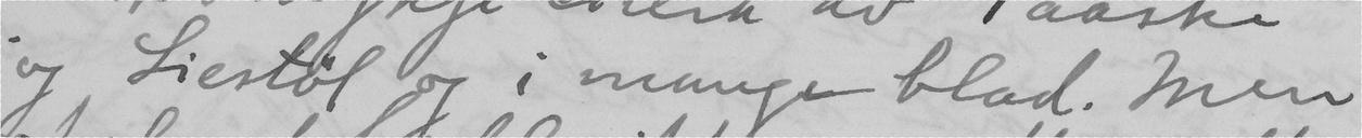og Liestöl og i mange blad. Men
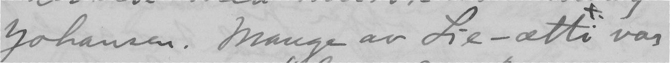Johansen. Mange av Lie-ætti –
